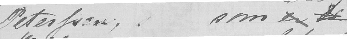Peterssen, som er dr
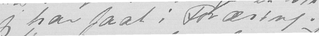jeg har faat i Foræring.
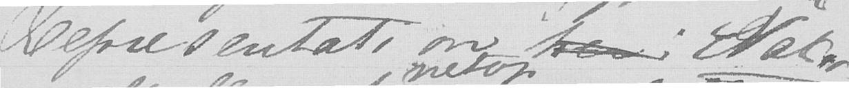Representation tren Natio-
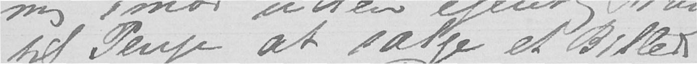til Penge at sælge et Billede
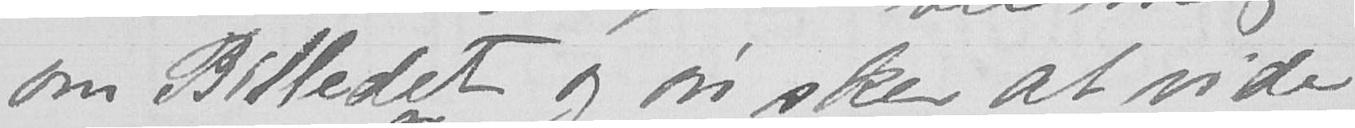om Billedet og ønsker at vide
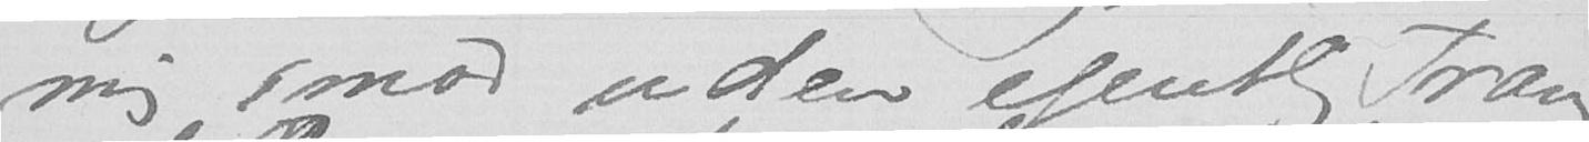mig imod uden egentlig Trang
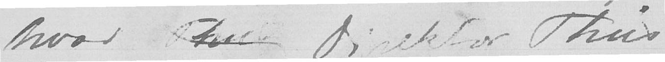hvad Thiis Direktør Thiis
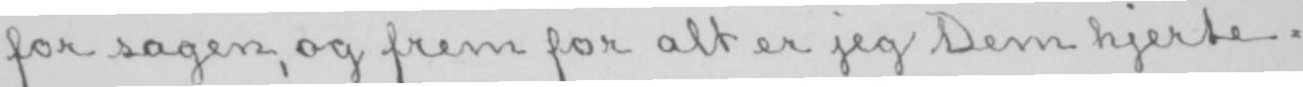for sagen, og frem for alt er jeg Dem hjerte-
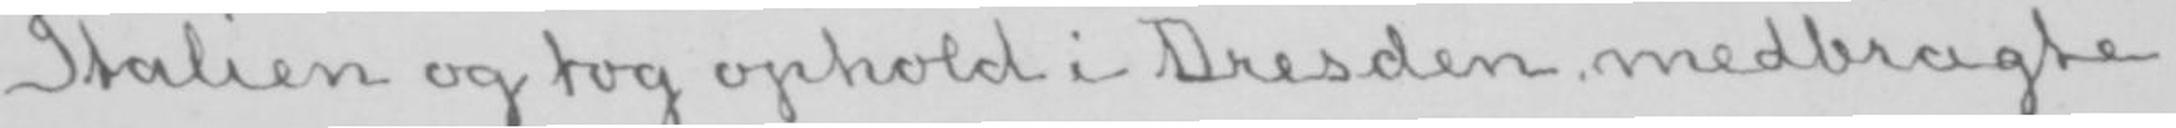Italien og tog ophold i Dresden medbragte
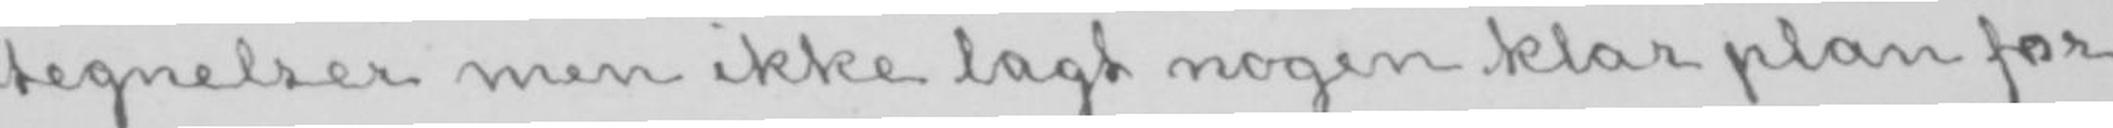tegnelser men ikke lagt nogen klar plan for
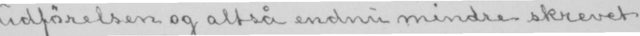udførelsen og altså endnu mindre skrevet
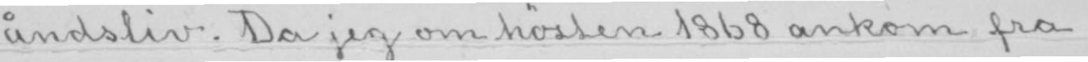åndsliv. Da jeg om høsten 1868 ankom fra
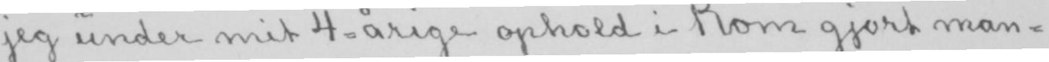jeg under mit 4-årige ophold i Rom gjort man-
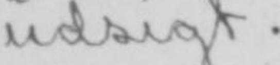udsigt.-
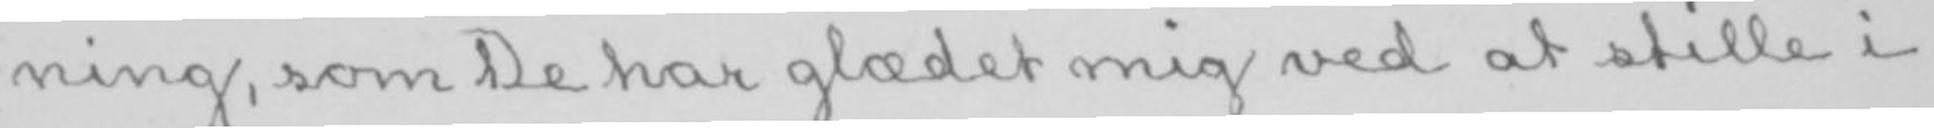ning, som De har glædet mig ved at stille i
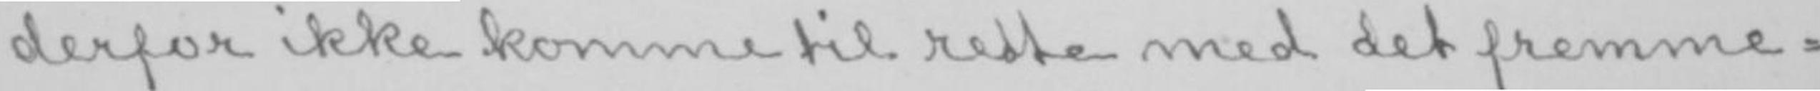derfor ikke komme til rette med det fremme-
